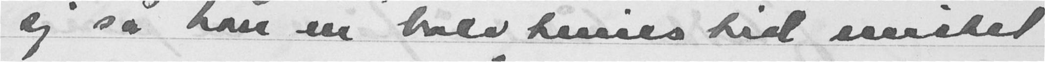sig så han en halv times tid mistet
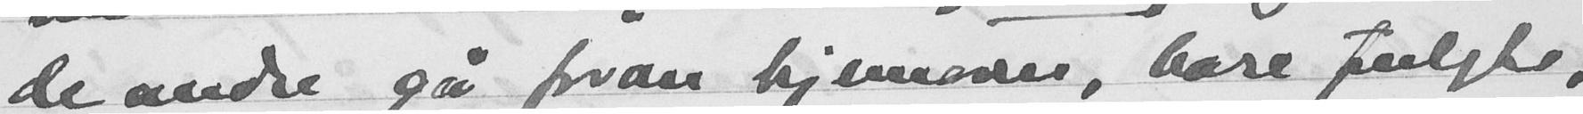de andre ga foran hjemover, bare fulgte,
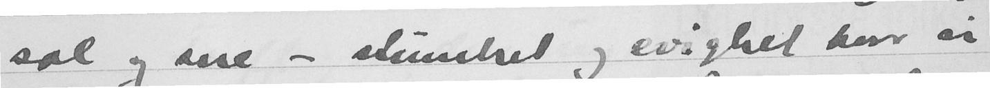sol og sne - stumhet og evighet hvor vi
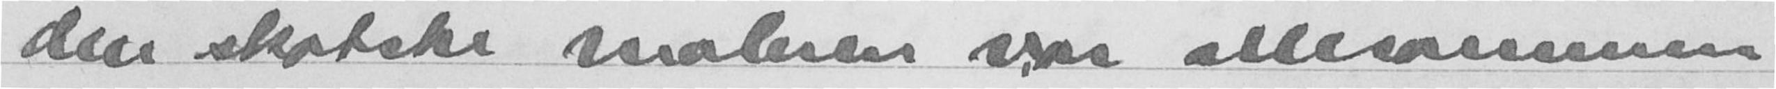den skotske maleren var allesammen
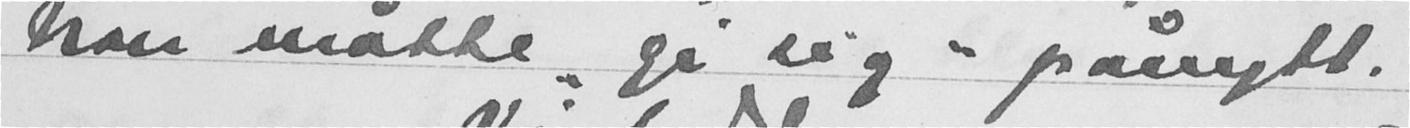han måtte gi sig pånytt.
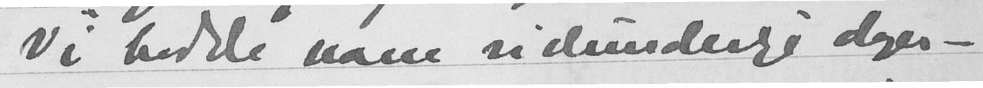Vi hadde masse vidunderlige dager -
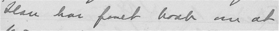Han har faaet haab om at
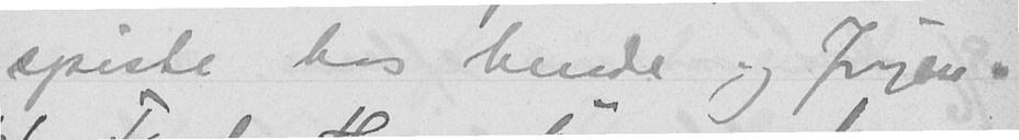spiste hos hende og Jørgen.
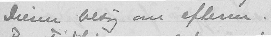Diesen besøg om efterm.
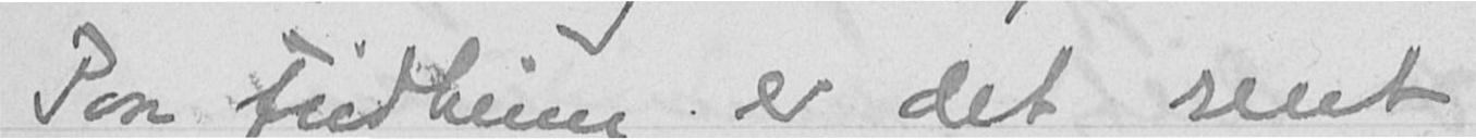Paa Fridheim er det rent
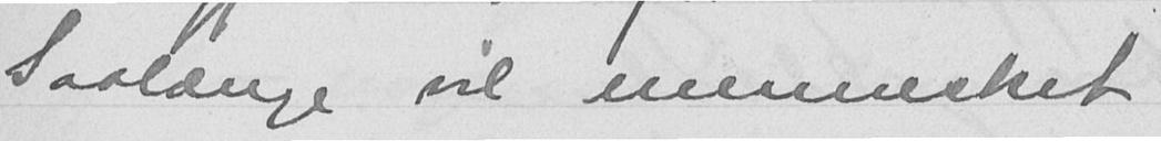Saalænge vil mennesket
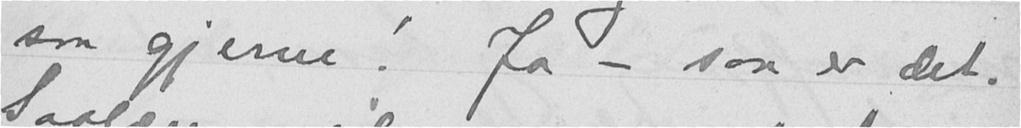saa gjerne! Ja - saa er det.
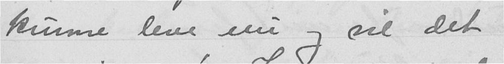kunne leve nu og vil det
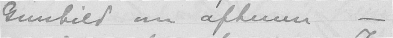Gunhild om aftenen -
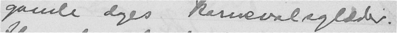gamle dages karnevalsglæder.
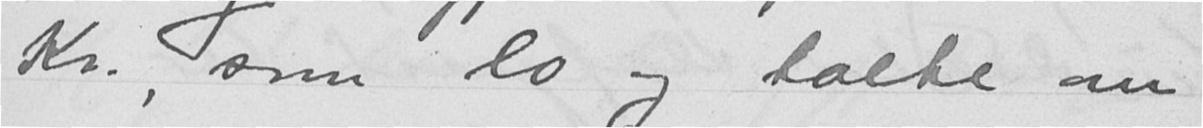Kr., som lo og talte om
